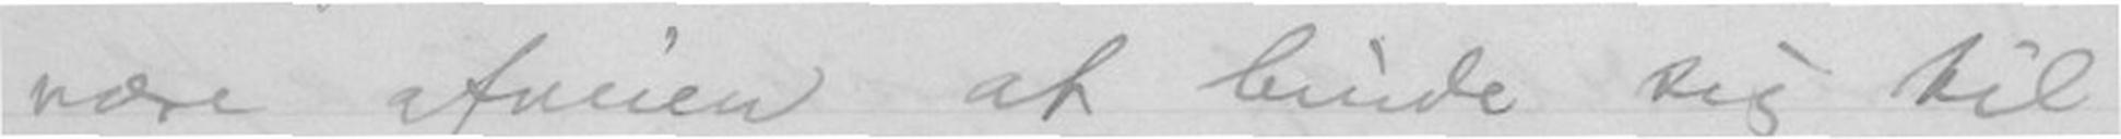være afveien at binde sig til
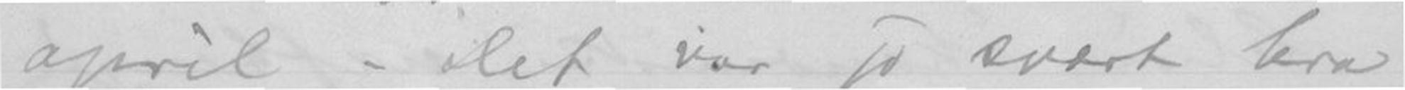april. Det var jo svært bra
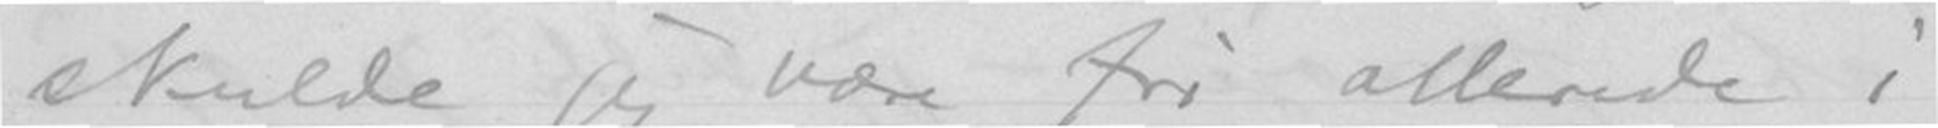skulde jeg være fri allerede i
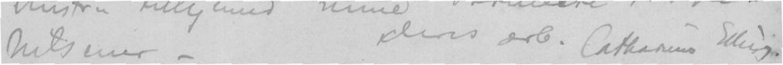hilsener -Deres ærb. Catharinus Elling.
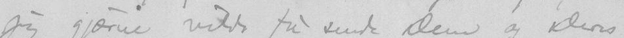jeg gjærne vilde få sende Dem og Deres
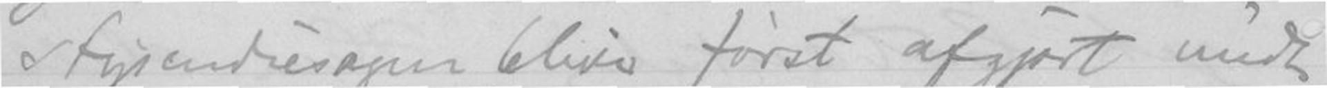stysendiesagen blive først opgjort midt
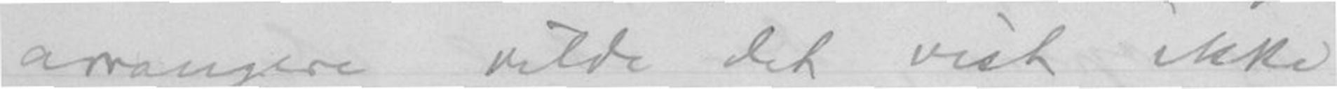arrangere vilde det vist ikke
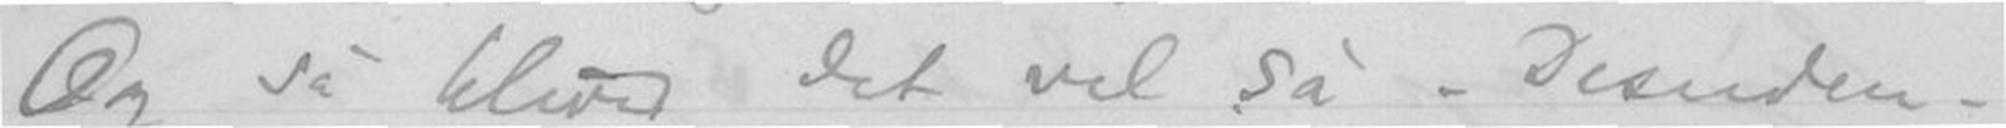Og så bliver det vel så. Desuden -
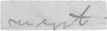meget.
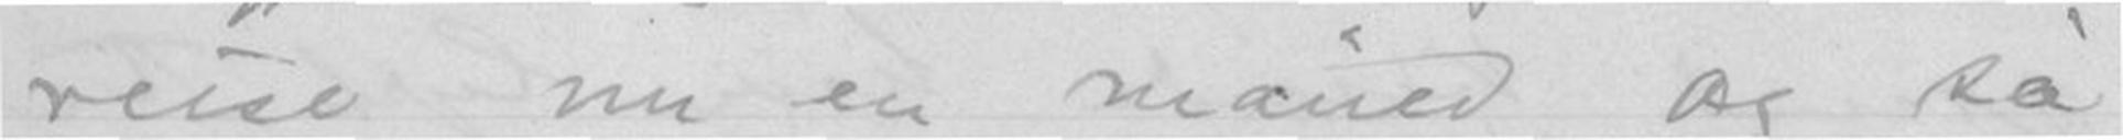reise nu en måned og så
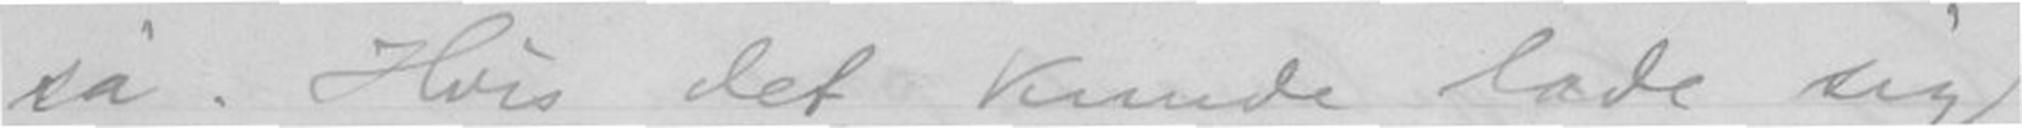så. Hvis det kunde lade sig
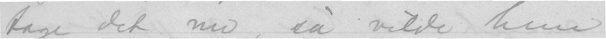tage det nu, så vilde hun
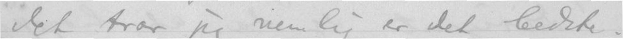det tror jeg nemlig er det bedste.
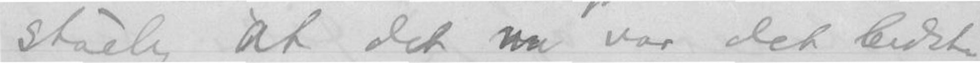ståelig at det nu var det bedste
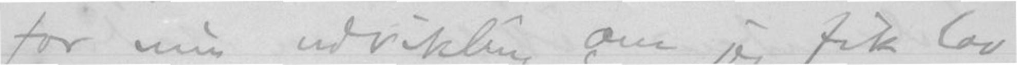for min udvikling om jeg fik lov
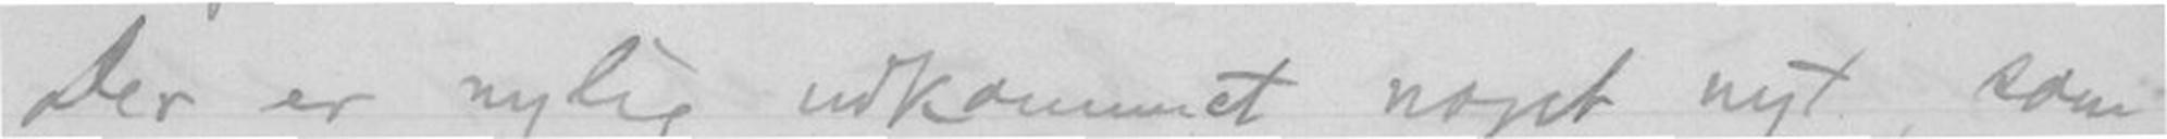Der er nylig udkommet noget nyt, som
